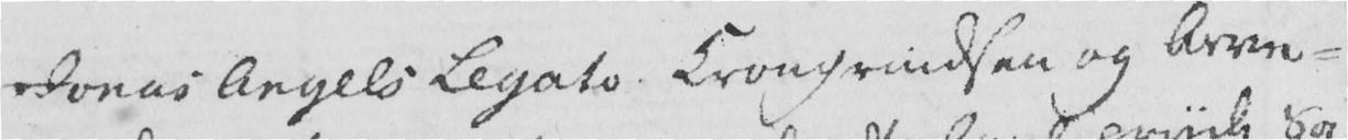Jonas Angels Legato. Kronprindsen og Arve-
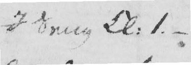I Seng Kl. 1. -
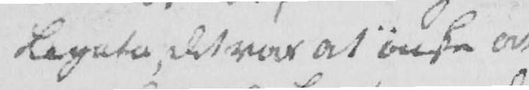Legata, det var at ønske at
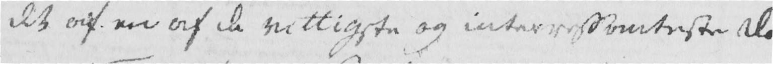det af en af de vittigste og interresanteste Da-
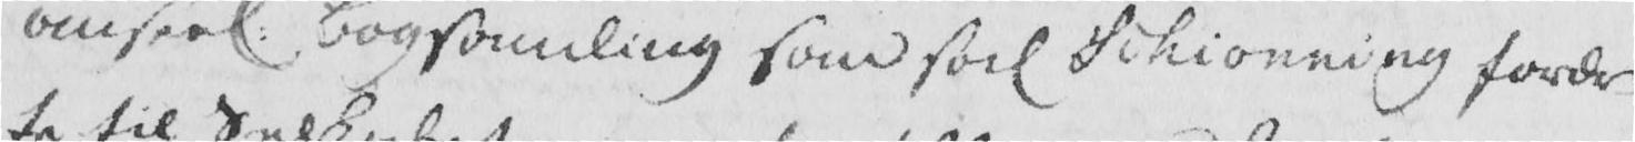anseel. Bogsamling som sal Schionning forære-
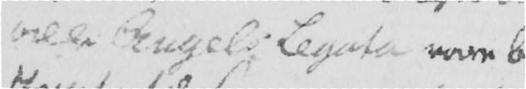alle Angels Legata vare be-
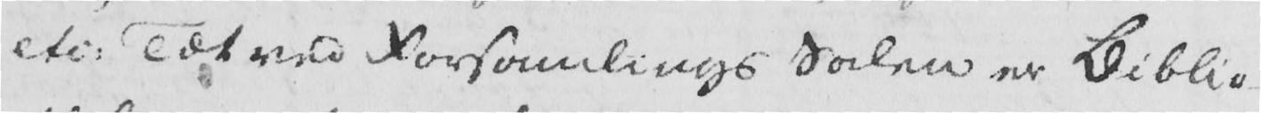etc. Tæt ved Forsamlings Salen er Biblio-
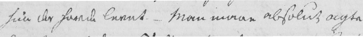hun der havde levet. - Man maae absolut agte
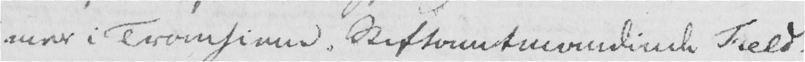mer i Tronhiem, Stiftamtmandinde Field-
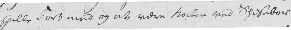spille Kort med og at være Naboe ved Spisebor-
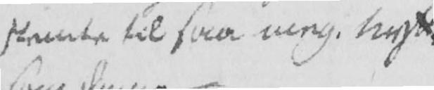stemte til saa meg. Nytte
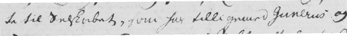te til Selskabet, som har tilligemed Gunerus og
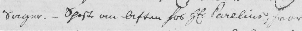Sager. - Spiste om Aften hos Hr. Parelius, hvor
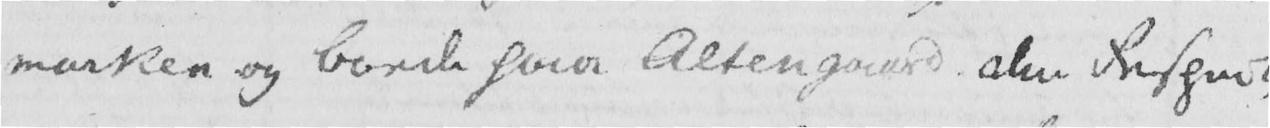marken og boede paa Altengaard. Den Respect
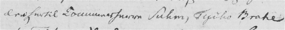len. Hertil Kammerherre Suhm, Tycho Brahe
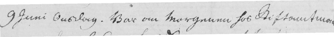9 Juni Onsdag. Var om Morgenen hos Stiftamtman-
